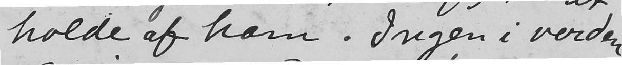holde af ham. Ingen i verden
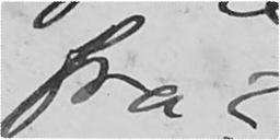fra?
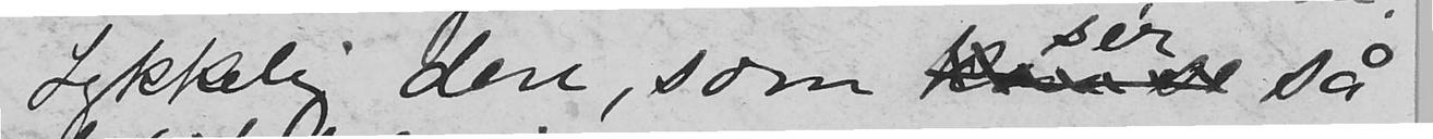Lykkelig den, som se'r så
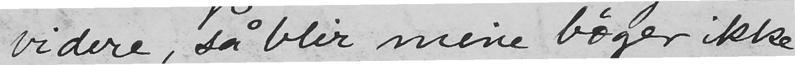videre, så blir mine bøger ikke
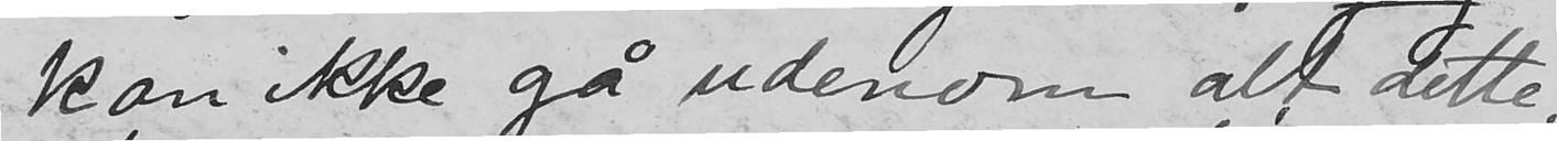kan ikke gå udenom alt dette.
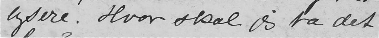lysere. Hvor skal jeg ta det
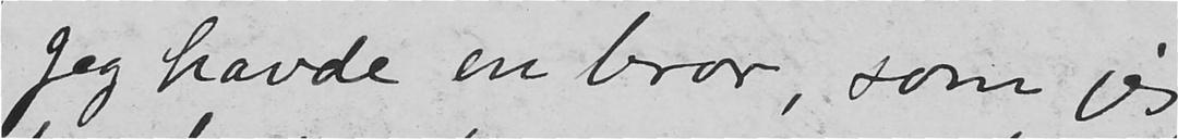Jeg havde en bror, som jeg
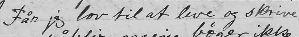Får jeg lov til at leve, og skrive
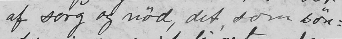af sorg og nød, det som søn-
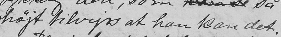højt tilvejrs at han kan det.
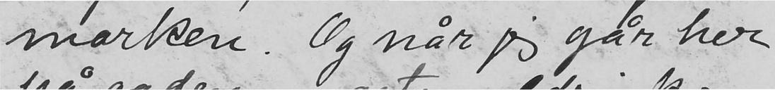marken. Og når jeg går her
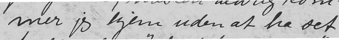mer jeg hjem uden at ha set
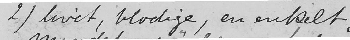2) livet, blodige, en enkelt
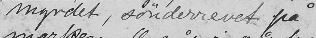myrdet, sønderrevet på
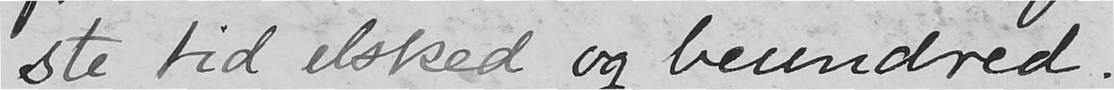ste tid elsked og beundred.
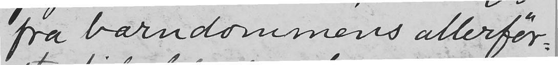fra barndommens allerfør-
